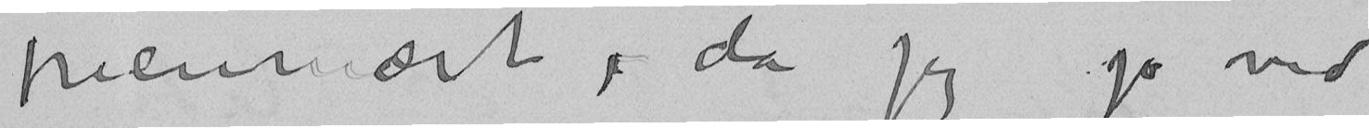pecuniært, da jeg jo ved
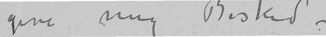give mig Besked –
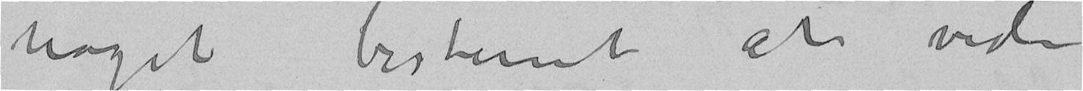noget bestemt at vide
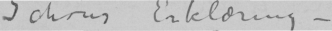Schous Erklæring –
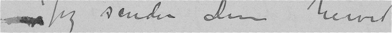Jeg sender Dem herved
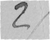2)
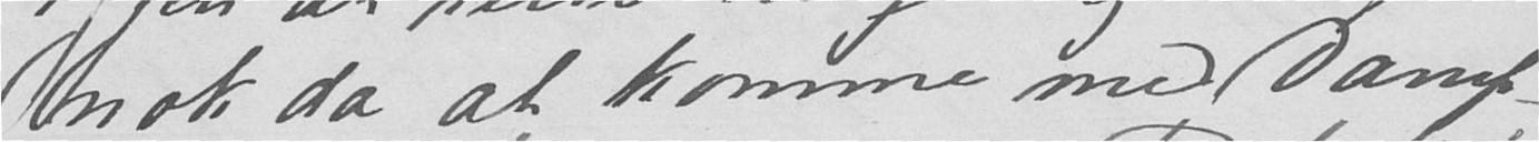nok da at komme med Damp-
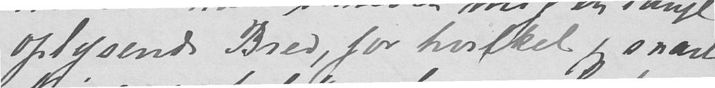oplysende Brev, for hvilket jeg snart
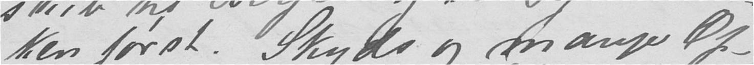ken først. Skyds og mange Op-
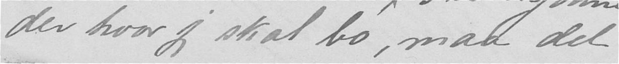der hvor jeg skal bo, maa det
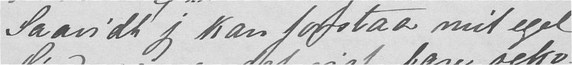Saavidt jeg kan forstaa mit eget
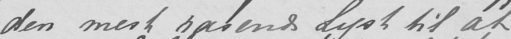den mest rasende Lyst til at
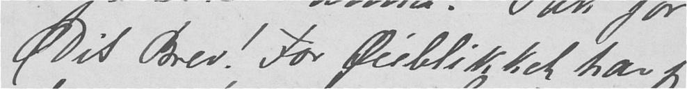Dit Brev! For Øieblikket har jeg
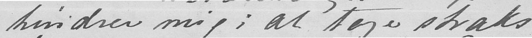hindrer mig i at tage straks
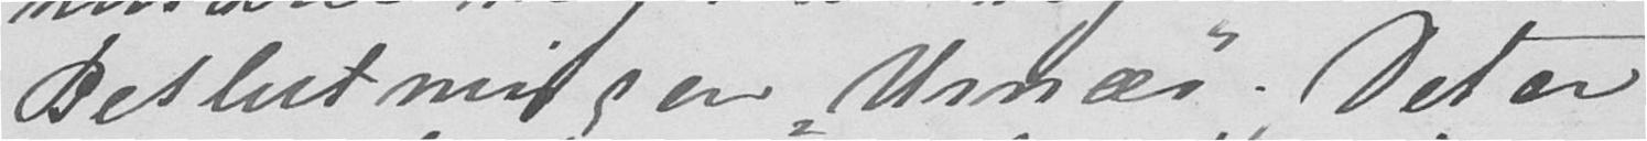Beslutningen "Urnæs". Det er
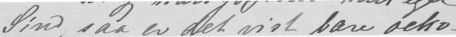Sind, saa er det vist bare oeko-
